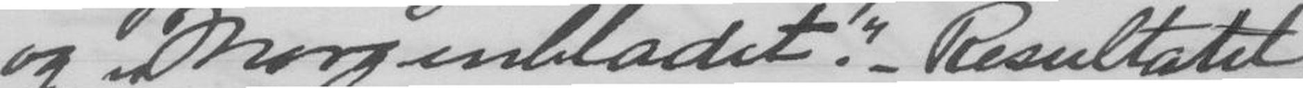og "Morgenbladet." _ Resultat
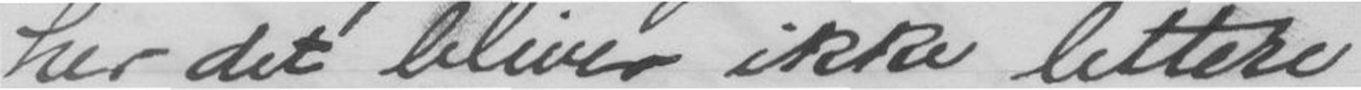her det bliver ikke lettere
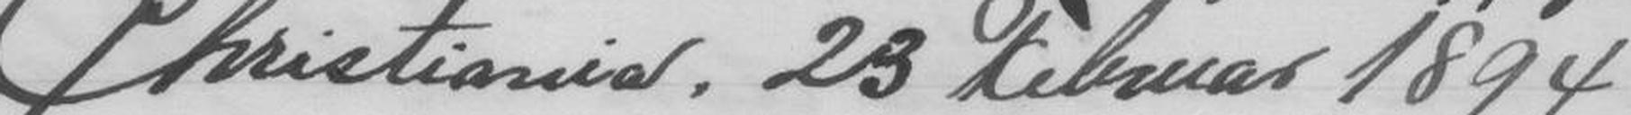Christiania. 23 Februar 1894.
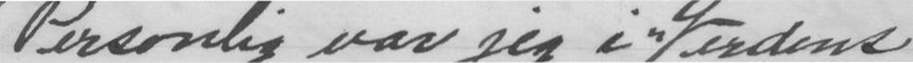Personlig var jeg i "Verdens
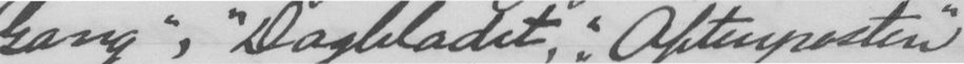Gang", "Dagbladet," "Aftenposten"
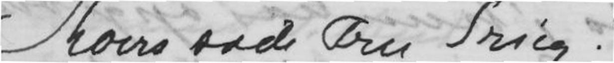Kaere søde Fru Grieg!
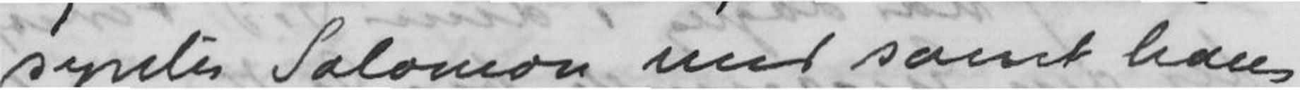syntes Salomon med samt hans
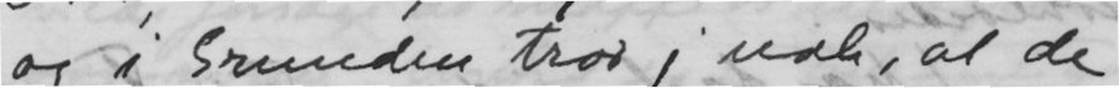og i Grunden tror j nok, at de
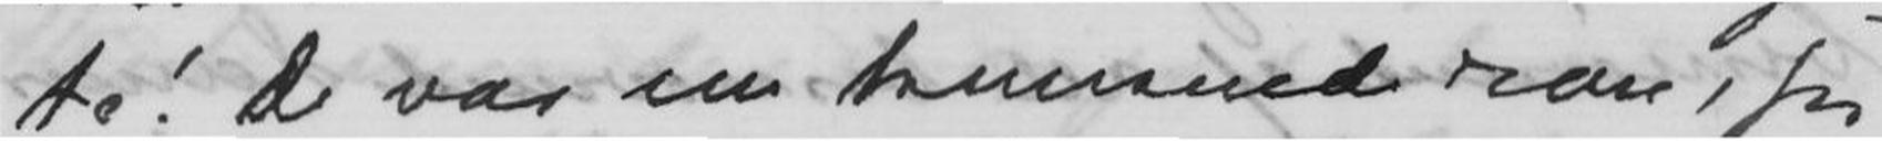te! De var en knusende rase, for
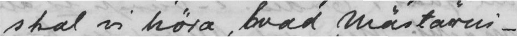skal vi höra, hvad Mästarin-
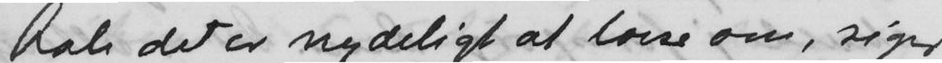Aah det er nydeligt at laese om, siger
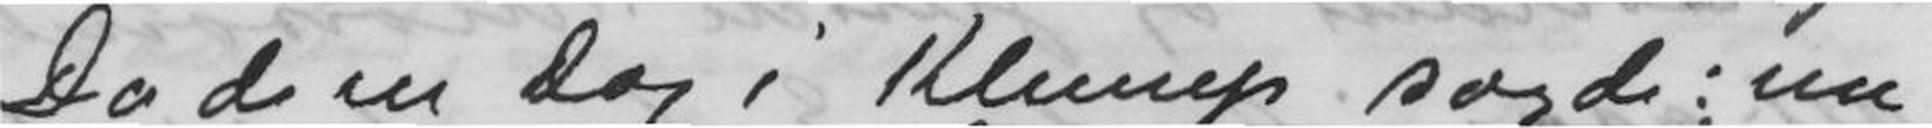Da de en Dag i Klump sagde: nu
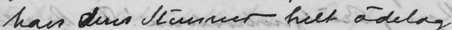have Deres Stunner helt ødelag-
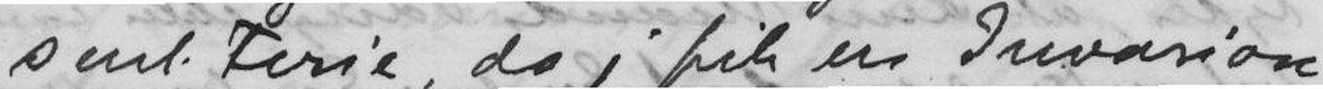sant Ferie, da j fik en Invasion
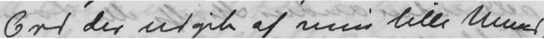Ord der udgik af min lille Mund,
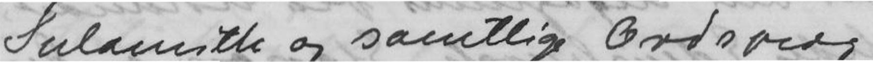Sulamith og samtlige Ordsprog
